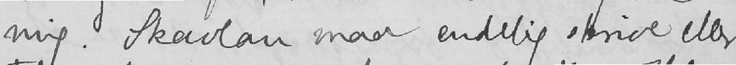mig. Skavlan maa endelig skrive eller
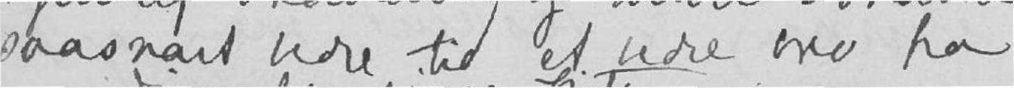saasnart bedre tid et bedre brev fra
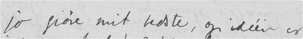jo gjøre mit bedste, og idéen er
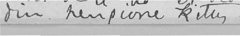din hengivne Kitty.
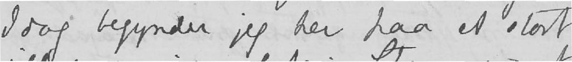Idag begynder jeg her paa et stort
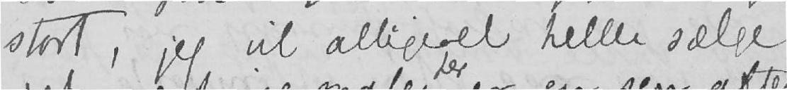stort, jeg vil alligevel heller sælge
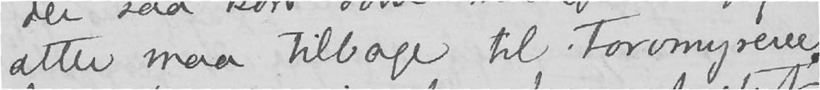atter maa tilbage til torvmyrene.
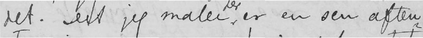det. Det jeg maler her er en sen aften-
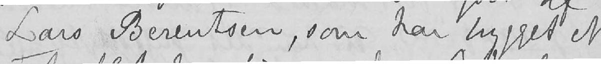Lars Berentsen, som har bygget et
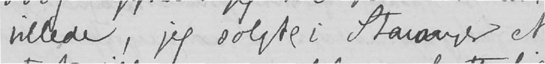billede, jeg solgte i Stavanger et
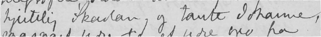hjertelig Skavlan og tante Johanne,
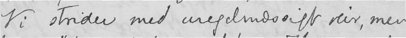Vi strider med uregelmæssigt veir, men
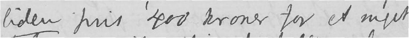liden pris 400 kroner for et meget
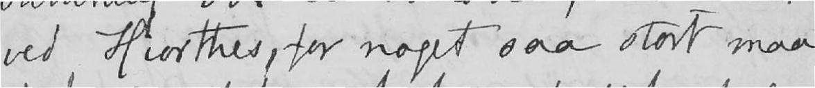ved Hiorthes, for noget saa stort maa
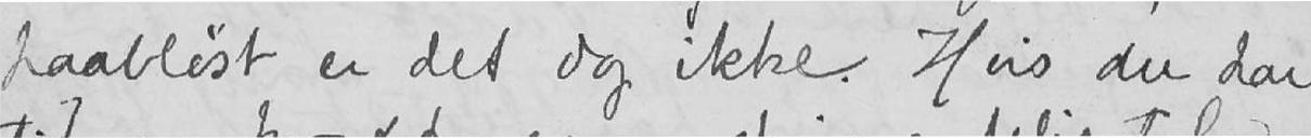haabløst er det dog ikke. Hvis du har
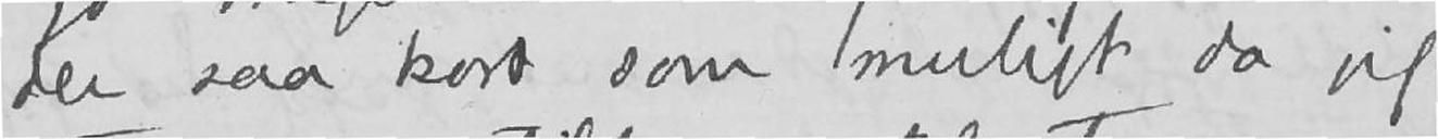der saa kort som muligt da jeg
